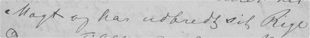Magt og har udbredt sit Rige.
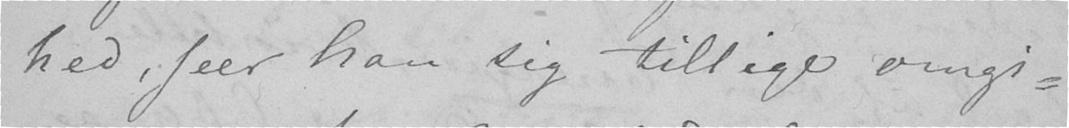hed, seer han sig tillige omgi-
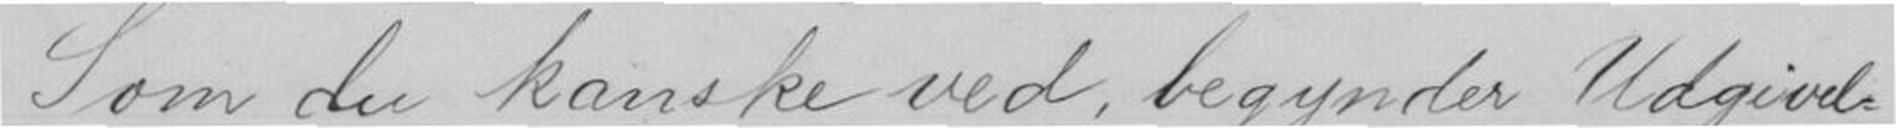Som du kanske ved, begynder Udgivel-
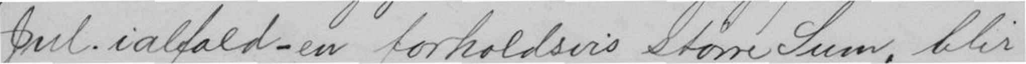Jul ialfald- en forholdsvis større Sum, blir
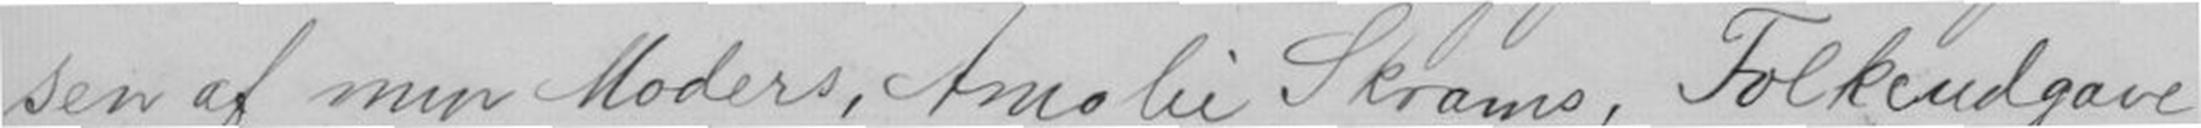sen af min Moders, Amalie Skrams, Folkeudgave
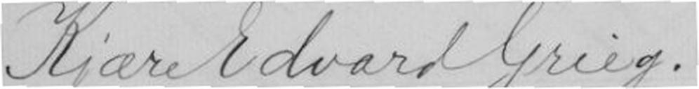Kjære Edvard Grieg !
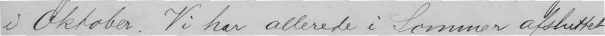i Oktober. Vi har allerede i Sommer afsluttet
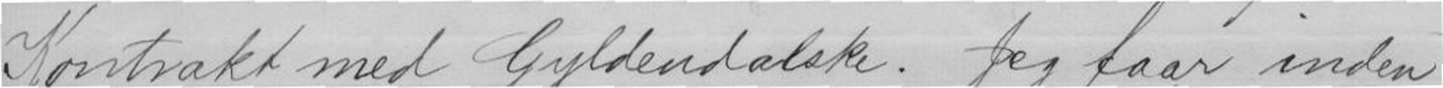Kontrakt med Gyldendalske. Jeg faar inden
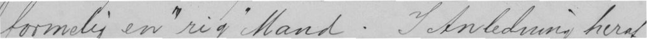formelig en "rig Mand". I Anledning heraf
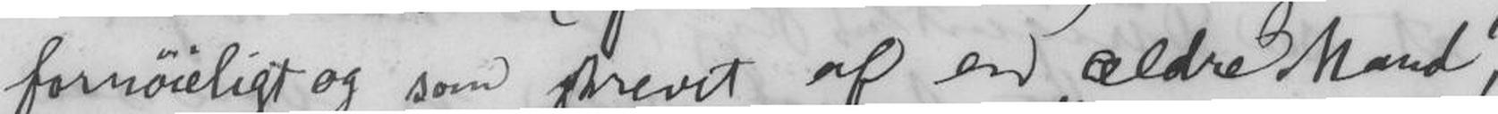fornøieligt og som skrevet af en ældre Mand,
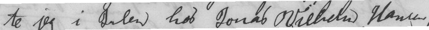te jeg i Julen hos Jonas Wilhelm Hansen,
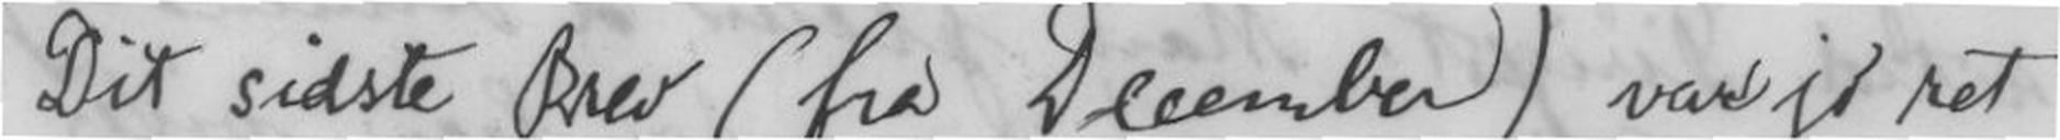Dit sidste Brev (fra December) var jo ret
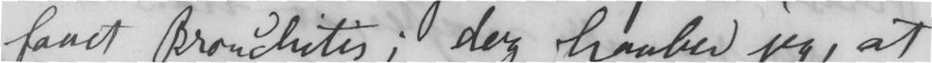faaet Bronchitis; dog haaber jeg, at
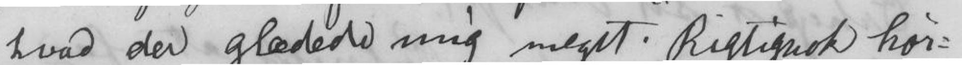hvad der glædede mig meget. Rigtignok hør-
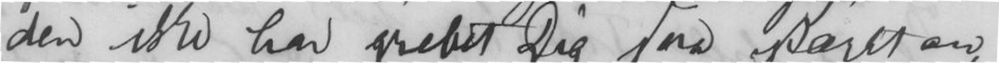den ikke har grebet Dig saa stærkt an,
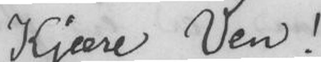Kjære Ven!
